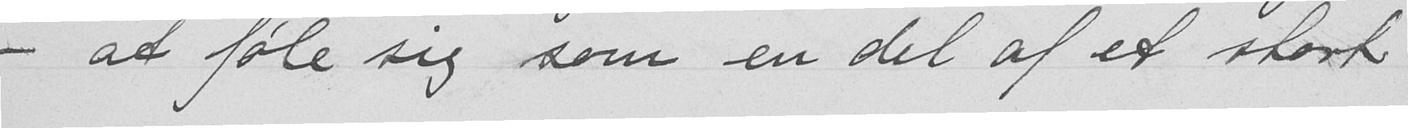- at føle sig som en del af et stort
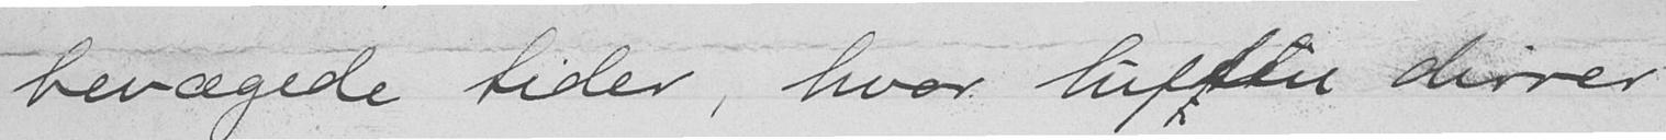bevægede tider, hvor luften dirrer
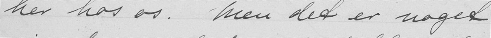her hos os. Men det er noget
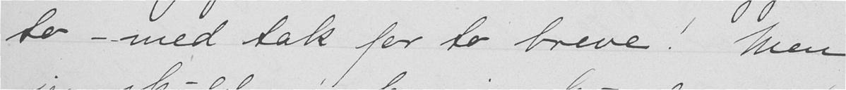to - med tak for to breve! Men
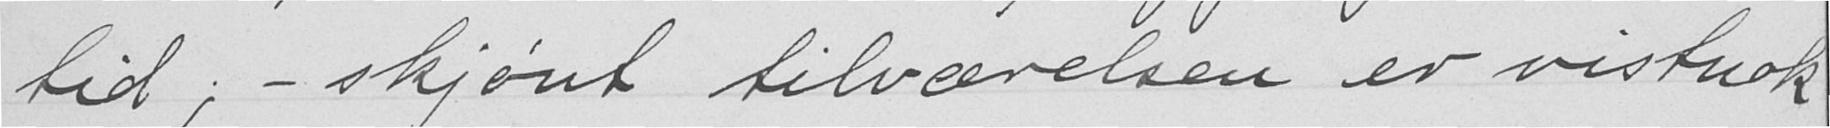tid, - skjønt tilværelsen er vistnok
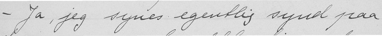- Ja, jeg synes egentlig synd paa
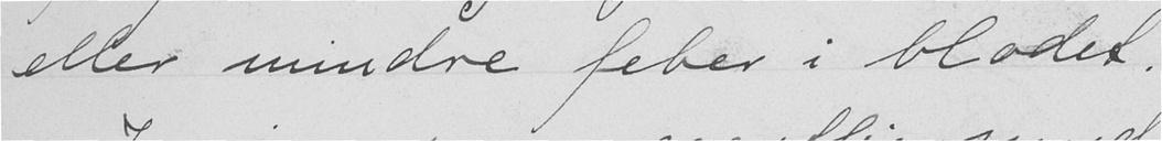eller mindre feber i blodet.
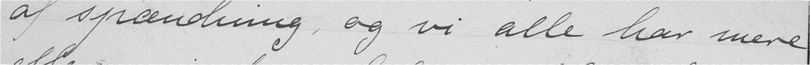af spændning, og vi alle har mere
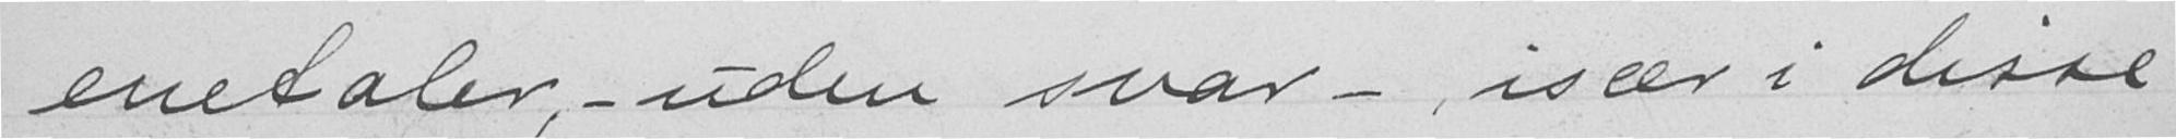enetaler, - uden svar -, især i disse
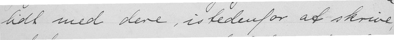lidt med dere, istedenfor at skrive,
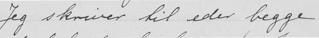Jeg skriver til eder begge
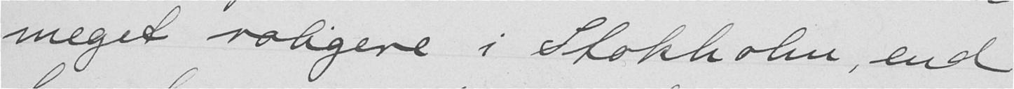meget roligere i Stokholm, end
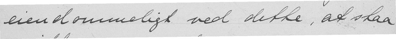eiendommeligt ved dette, at staa
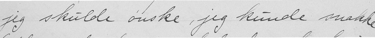jeg skulde ønske, jeg kunde snakke
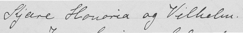Kjære Honoria og Vilhelm!
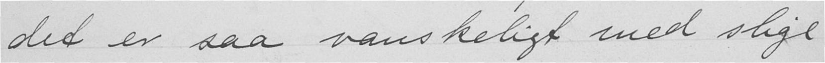- det er saa vanskeligt med slige
